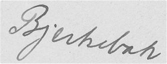Bjerkebæk
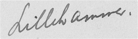Lillehammer,
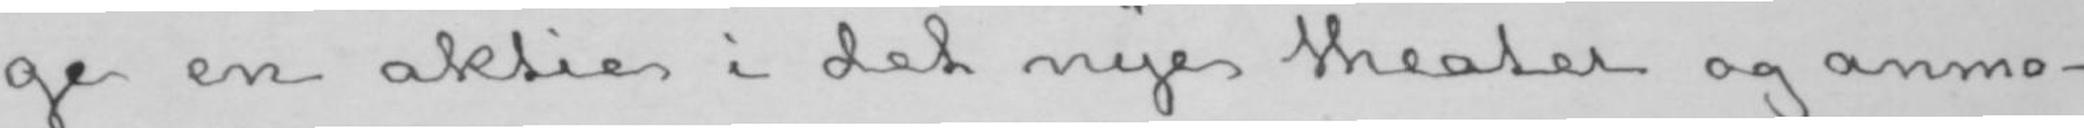ge en aktie i det nye theater og anmo-
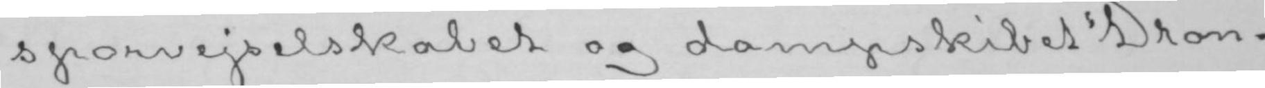sporvejselskabet og dampskibet «Dron-
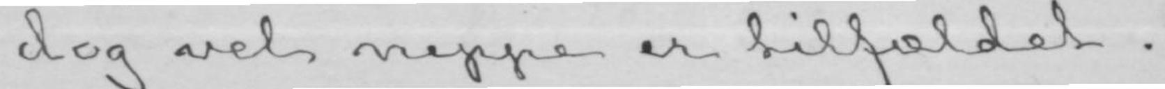dog vel neppe er tilfældet.
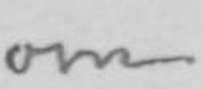om
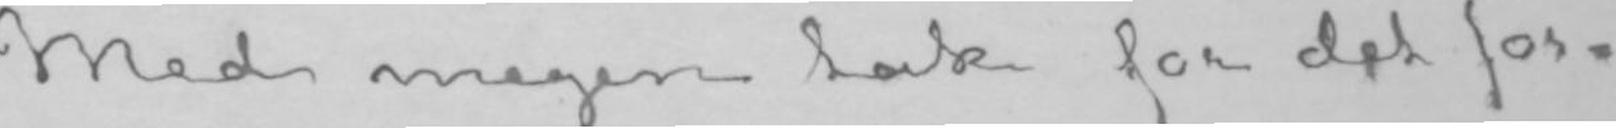Med megen tak for det for-
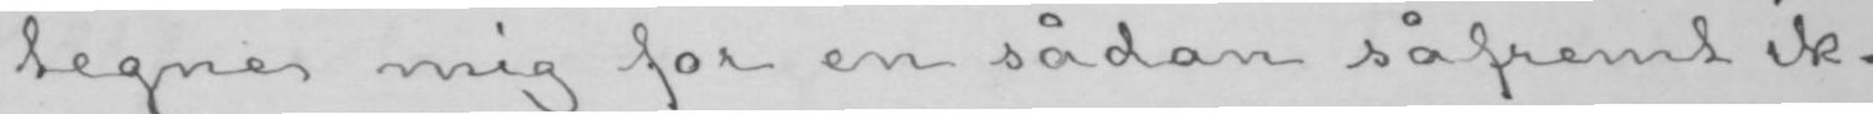tegne mig for en sådan såfremt ik-
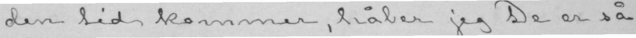den tid kommer, håber jeg De er så
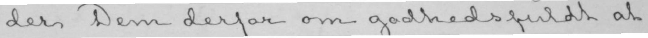der Dem derfor om godhetsfuldt at
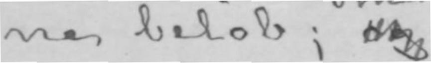ne beløb; og
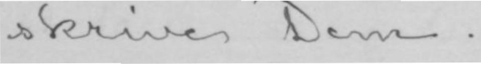skrive Dem.
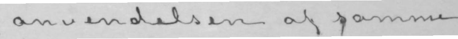anvendelsen af samme
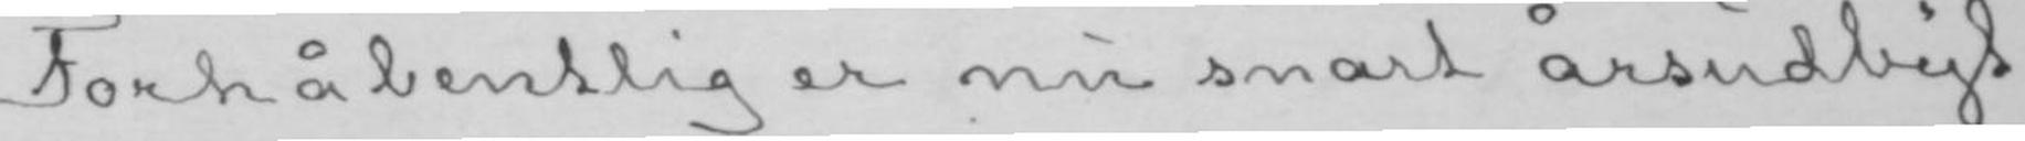Forhåbentlig er nu snart årsudbyt-
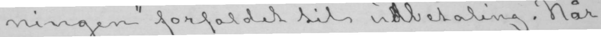ningen» forfaldet til udbetaling. Når
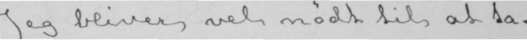Jeg bliver vel nødt til at ta-
